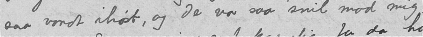saa vondt ihøst, og De var saa snil mod mig,
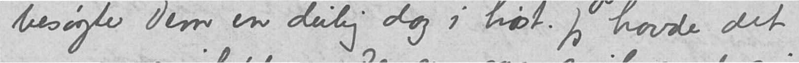besøgte Dem en deilig dag i høst. Jeg havde det
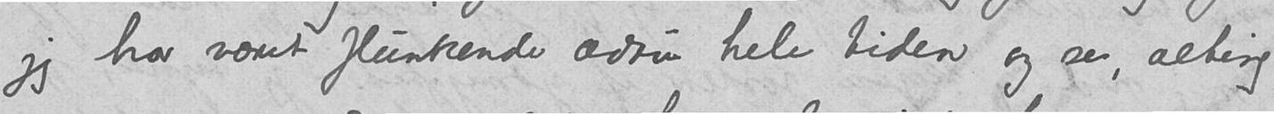jeg har været flunkende ædru hele tiden og ser, alting
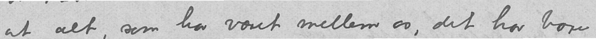at alt som har været mellem os, det har bare
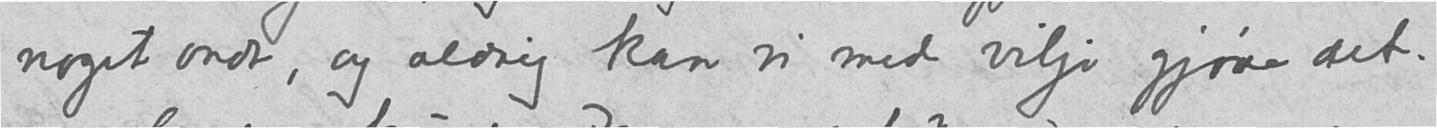noget ondt, og aldrig kan vi med vilje gjøre det.
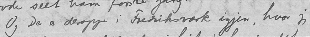Og De er deroppe i Fredriksværk igjen, hvor jeg
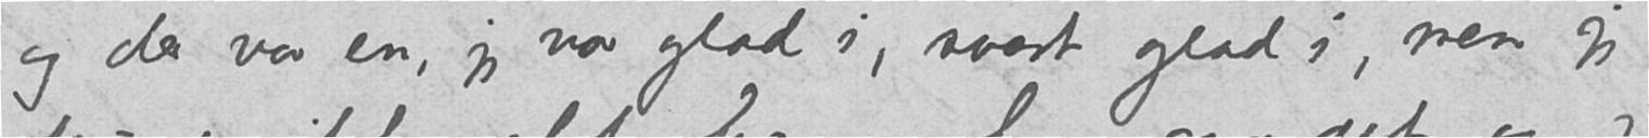og der var en, jeg var glad i, svært glad i, men jeg
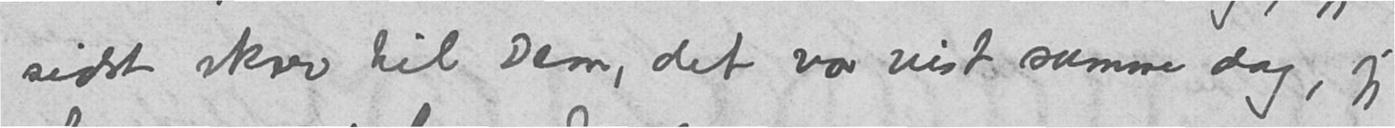sidst skrev til Dem, det var vist samme dag, jeg
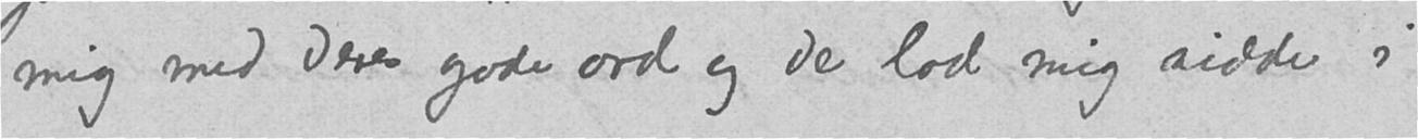mig med Deres gode ord og de lod mig sidde i
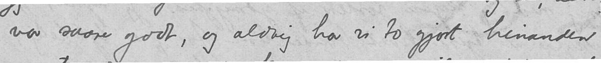var saare godt, og aldrig har vi to gjort hinanden
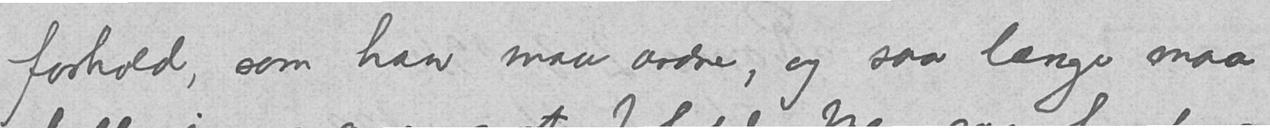forhold, som han maa ordne, og saa længe maa
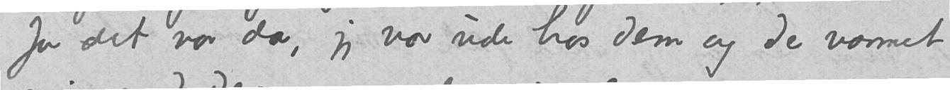for det var da, jeg var ude hos Dem og De varmet
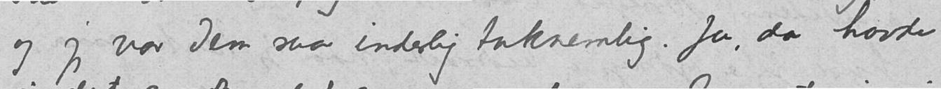og jeg var Dem saa inderlig taknemlig. Ja, da havde
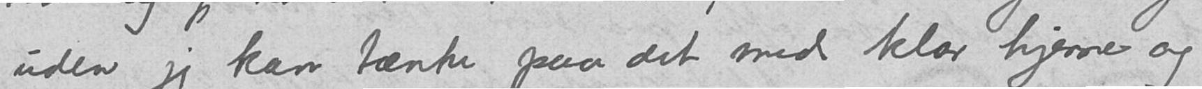uden jeg kan tænke paa det med klar hjerne og
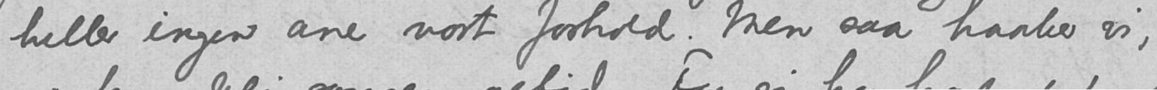heller ingen ane vort forhold. Men saa haaber vi,
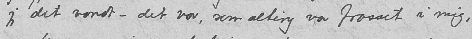jeg det vondt - det var, som alting var frosset i mig,
